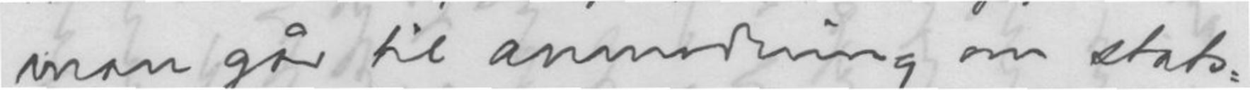man går til anmodning om stats-
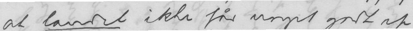at landet ikke får nogel godt af
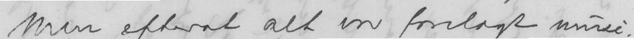Men efterat alt ere forelagt musi-
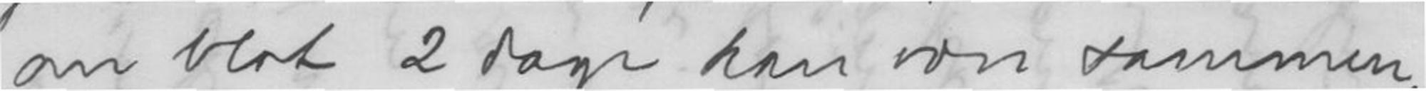om blot 2 dage kan være sammen,
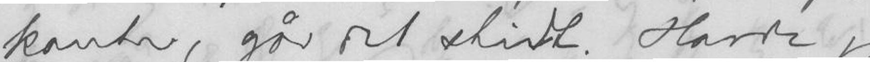kanter, går det skidt. Havde jeg
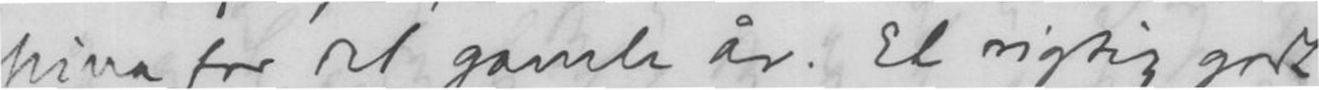Nina for det gamle år. Et rigtig godt
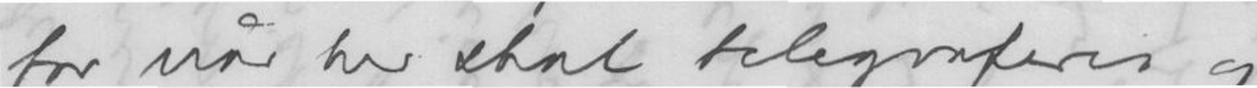for når her skal telegraferes og
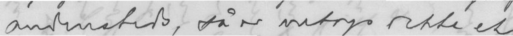andrensteds, så er netop dette et
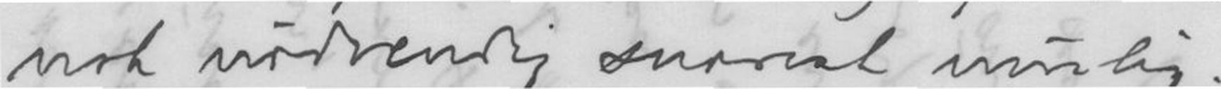nok nødvendig snarest mulig.
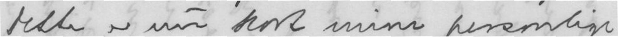Dette er nu kort mine persolige
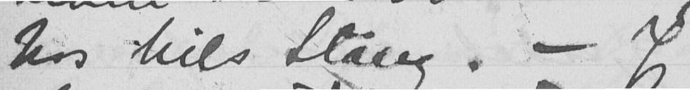hos Nils Stang. - Jeg
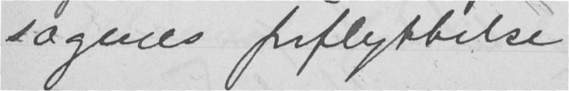sagenes forflyttelse
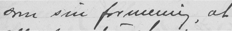som sin formening, at
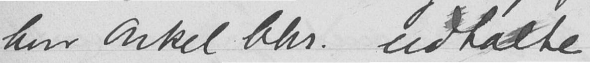hvor Onkel Chr. udtalte
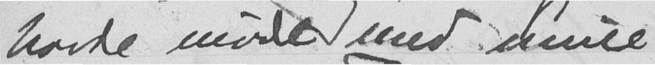havde møde med mine
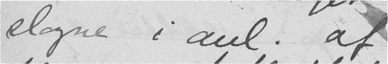slagne i anl. af
